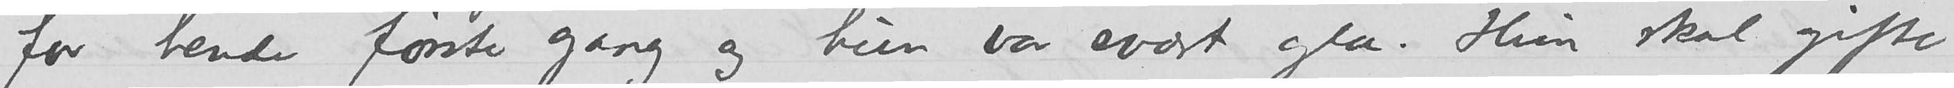for hende første gang og hun var svært gla. Hun skal gifte
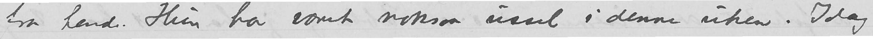fra hende. Hun har været noksaa ussel i denne uken. Idag
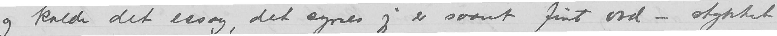og kalde det essay, det synes jeg er saant fint ord -stykket
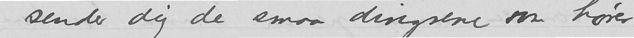Jeg sender dig de smaa dingsene som hører
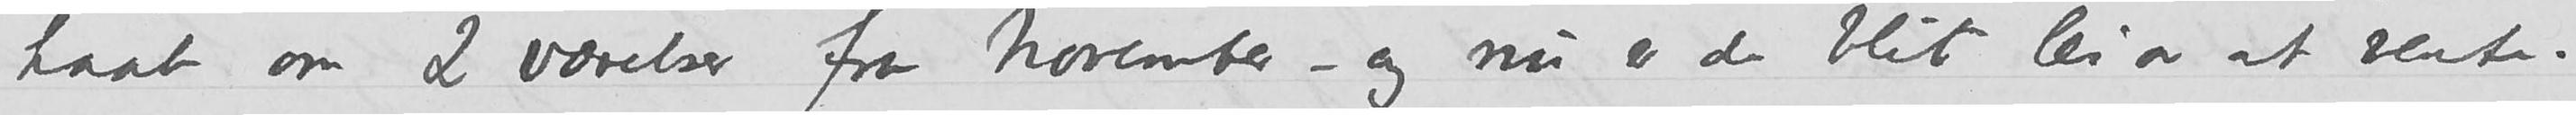haab om 2 værelser fra November -og nu er de blit lei av at vente.
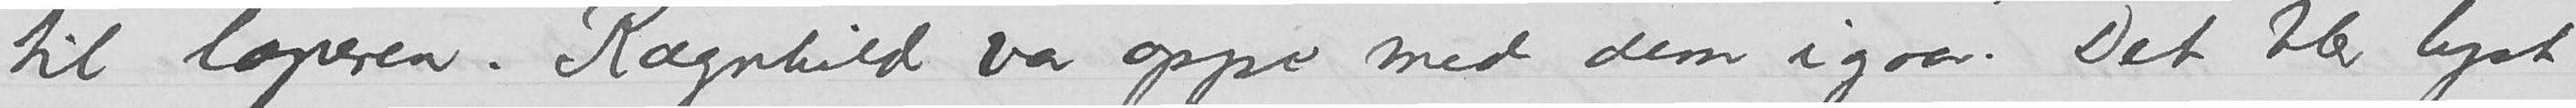til løperen. Ragnhild var oppe med dem igaar. Det blev lyst
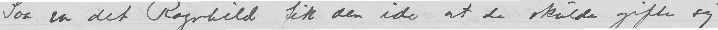Saa var det Ragnhild fik den ide at de skulde gifte sig
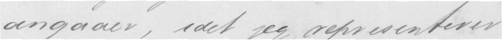angaaer, idet jeg representerer
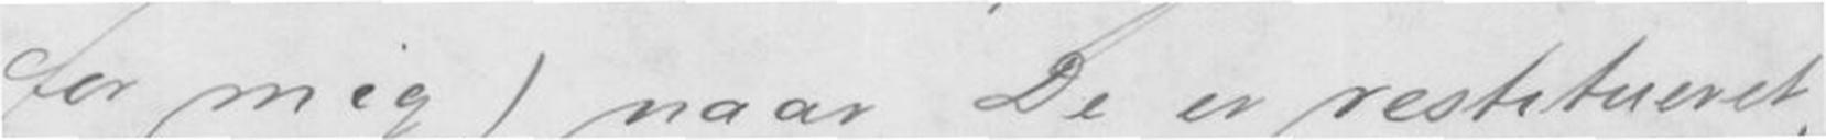for mig) naar De er restitueret,
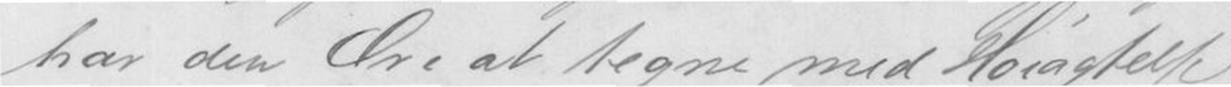har den Ære at tagne med Høiagtelse
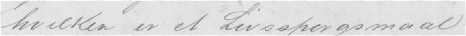(hvilket er et Livsspørgsmaal
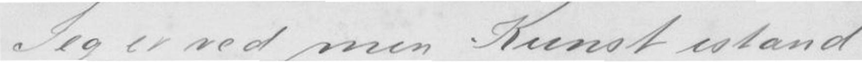Jeg er ved min Kunst istand
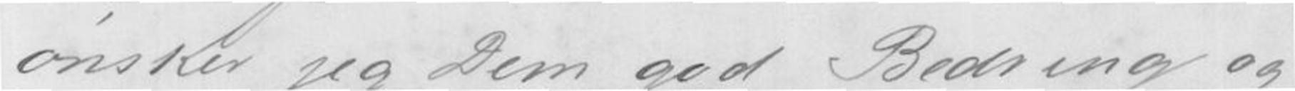ønsker jeg Den god Bedring og
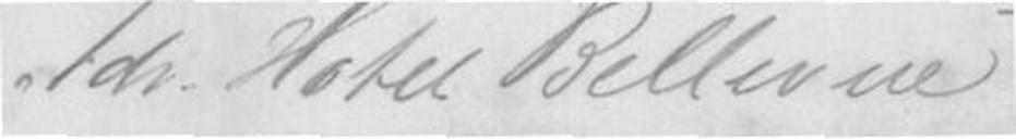Adr. Hotel Bellevue
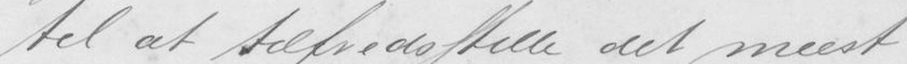til at Tilfredstille det meest
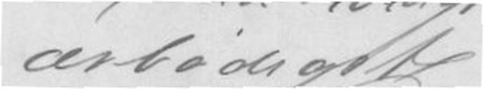Ærbødiget
